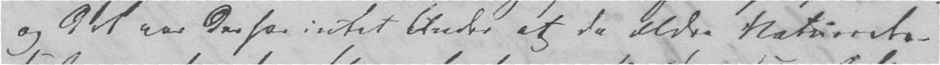og det var derfor intet Under at de ældre Naturrets-
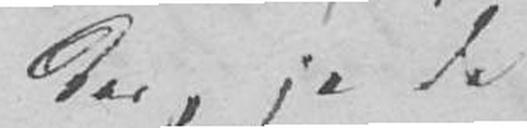der, ja de
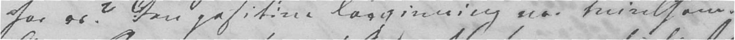hos os? Den positive Lovgivning var tvivlsom.
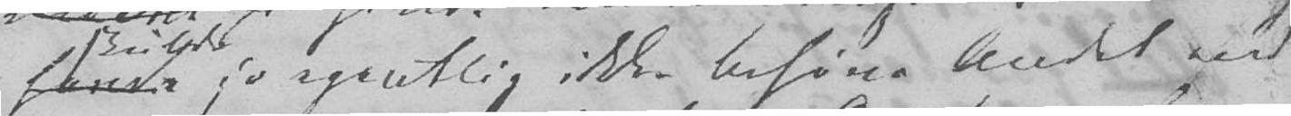jo egentlig ikke behøve Andet end
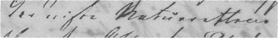der viste Naturrettens
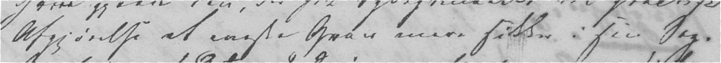Afgjørelse et eneste Gran mere sikker i sin Sag.
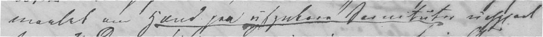maalet om Hævd paa usynbare Servituter uafgjort
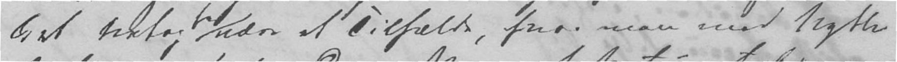det netop være et Tilfælde, hvor man med Nytte
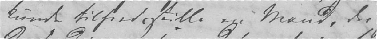kunde tilfredstille en Mand, der
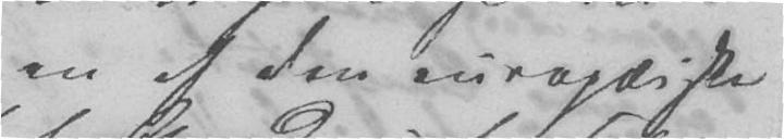en af den europæiske
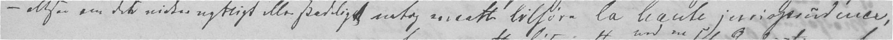- altsaa om det virker nyttigt eller skadeligt netop maatte tilhøre la haute jurisprudence,
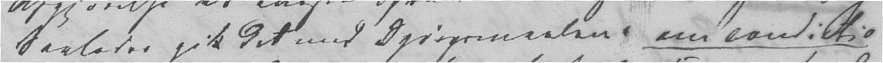Saaledes gik det med Spørgsmaalene om condictio
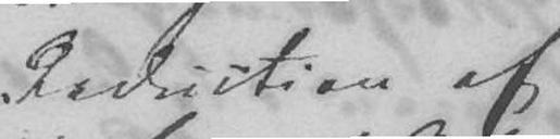Deduction af
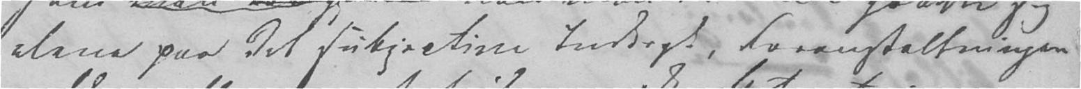alene paa det subjective Indtryk, Foranstaltningen
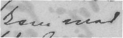kom med
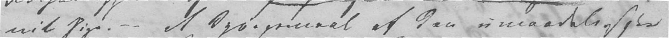vil sige - et Spørgsmaal af den umaadeligste
